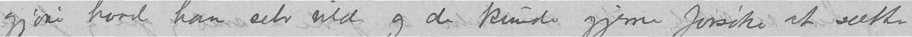gjøre hvad han selv vilde og de kunde gjerne forsøke at sætte
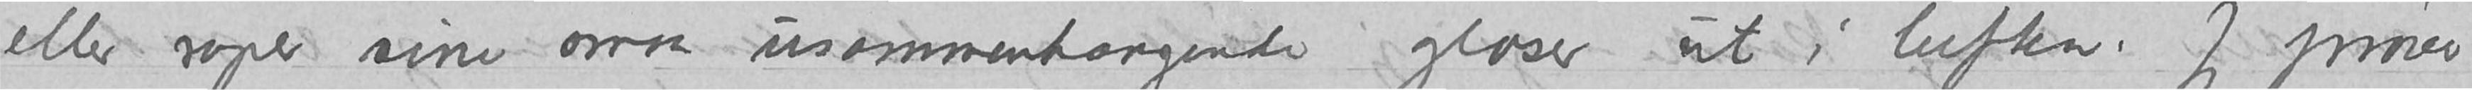eller roper sine arme usammenhængende gloser ut i luften. Jeg prøver
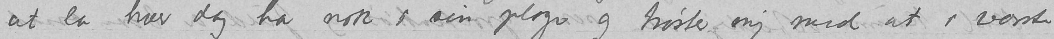at la hver dag ha nok av sin plage og trøster mig med at i værste
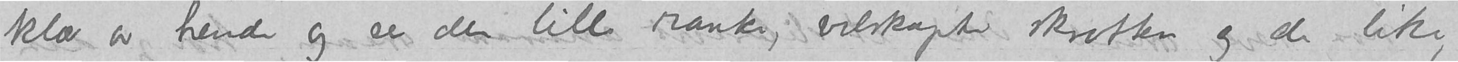klær av hende og ser den lille ranke, velskapte skrotten og de like,
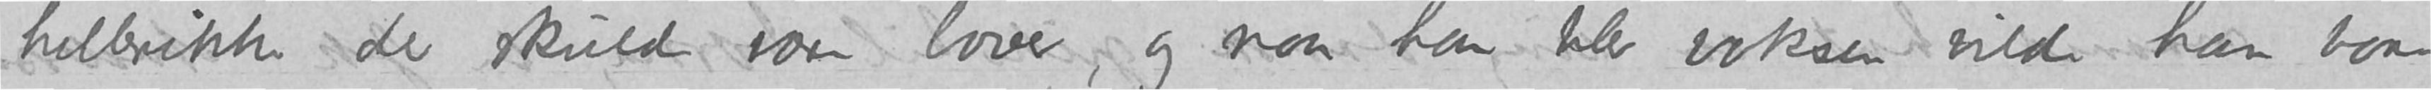hellerikke der skulde være lover, og naar han blev voksen vilde han bare
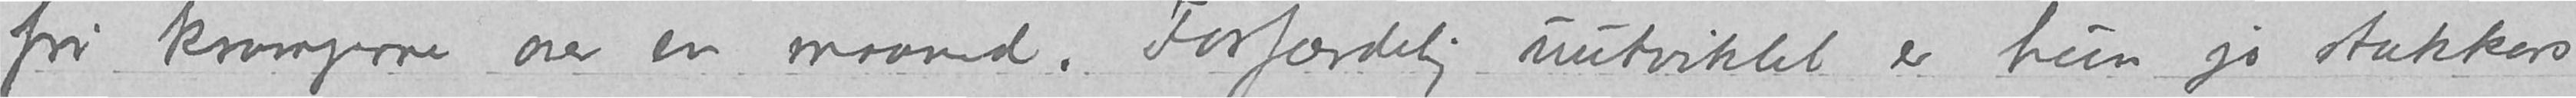fri kramperne over en maaned. Forfærdelig uutviklet er hun jo stakkars
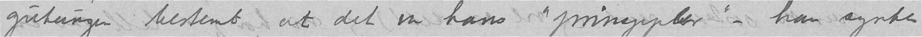gutungen bestemt, at det var hans »princypler» - han syntes
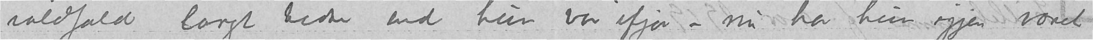ialfald langt bedre end hun var i fjor – nu har hun igjen været
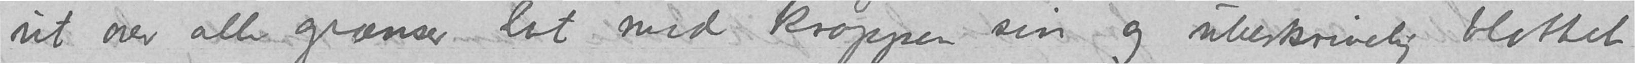ut over alle grænser lat med kroppen sin og ubeskrivelig blottet
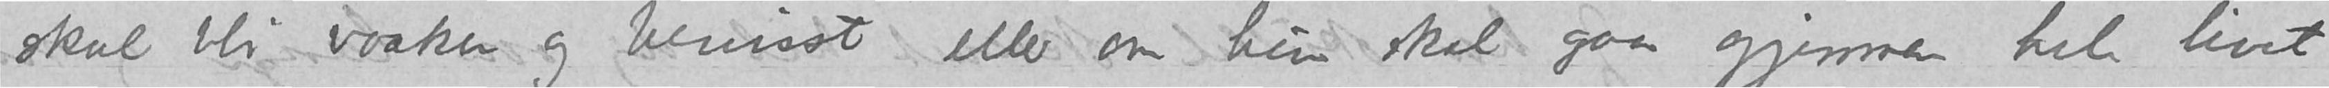skal bli vaaken og bevisst eller om hun skal gaa gjennem hele livet
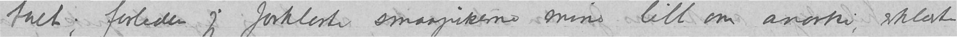talt; forleden jeg forklarte smaapikerne mine litt om anarki, erklærte
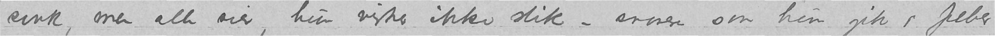vak, men alle sier, hun virker ikke slik – snarere som hun gik i feber.
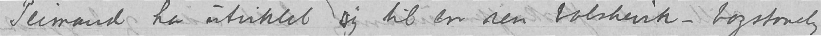Peimand har utviklet sig til en ren bolshevik – bogstavelig
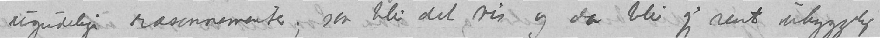ugudelige ræsonnementer; saa blir det ris og da blir jeg rent uhyggelig
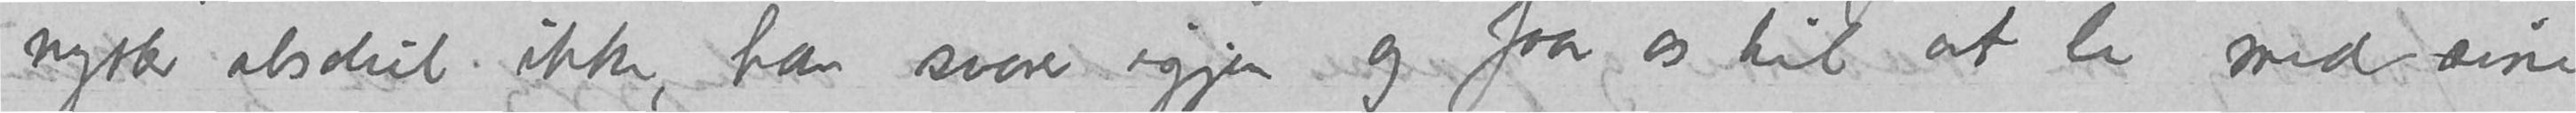nytter absolut ikke, han svarer igjen og faar os til at le med sine
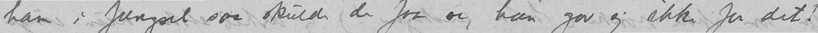ham i fængsel saa skulde de faa se, han gav sig ikke for det!
